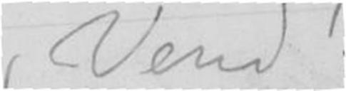(Vend!)
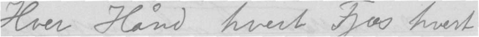Hver Hånd hvert Fjæs hvert
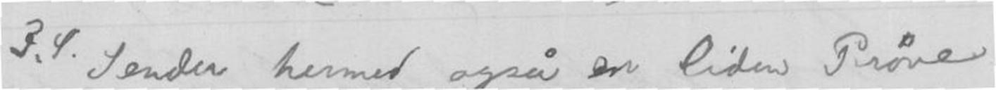P.S.Sender herved også en liden Prøve
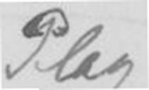Plag
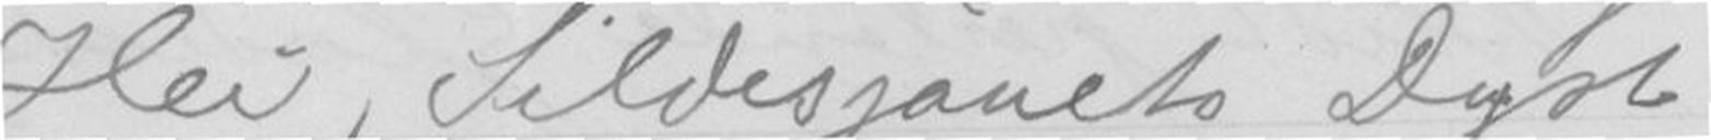Hei, Sildesjauets Dyst
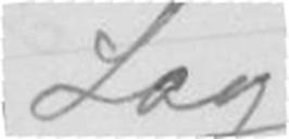Lag,
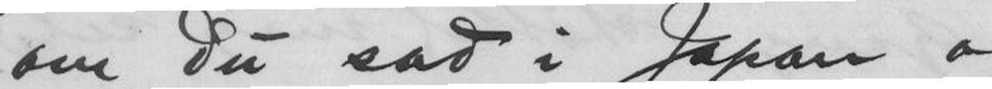om Du sad i Japan og
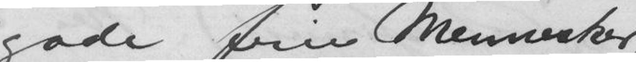af gode fine Mennesker,
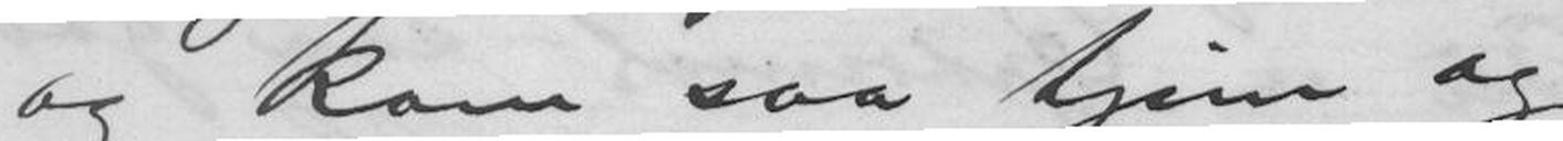og kom saa hjem og
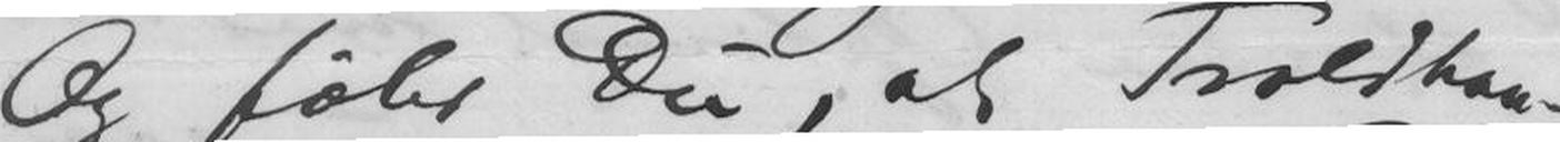Og føler Du, at Troldhau-
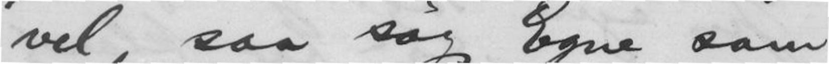vel, saa søg Egne som
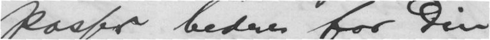passer bedre for Din
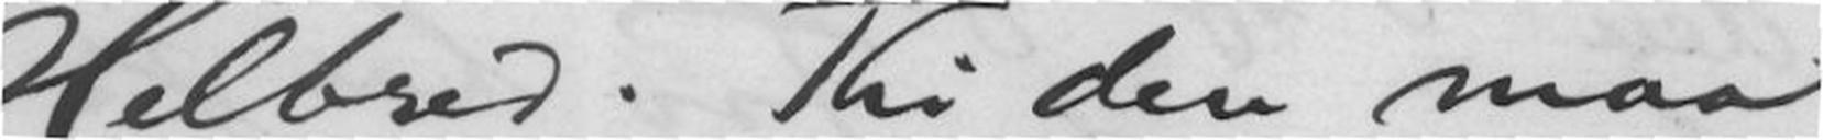Helbred. Thi den maa
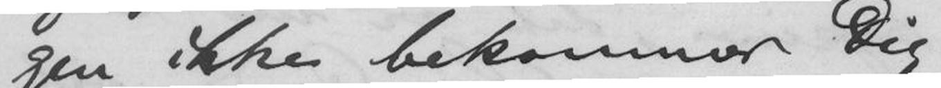gen ikke bekommer Dig
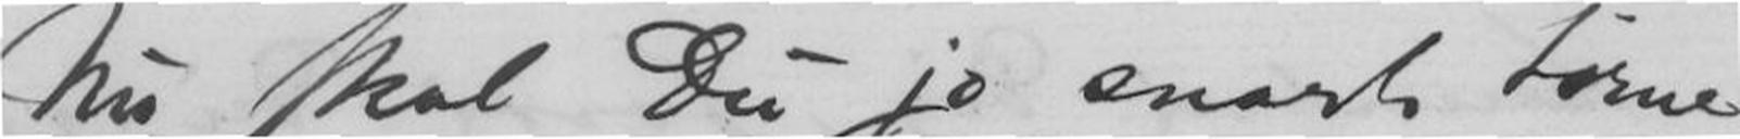Nu skal Du jo snart tørne
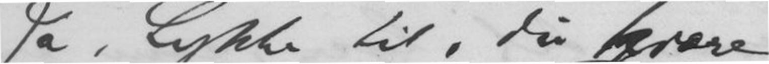Ja, Lykke til, du kjære
